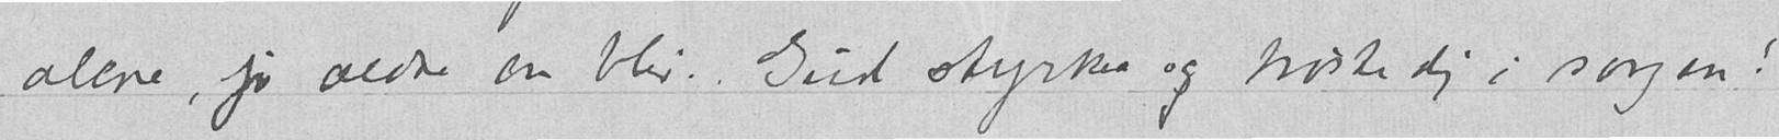alene, jo ældre en blir. Gud styrke og trøste dig i sorgen!
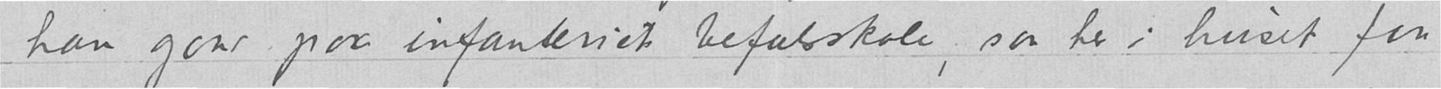han gaar paa infanteriets befalsskole, saa her i huset faar
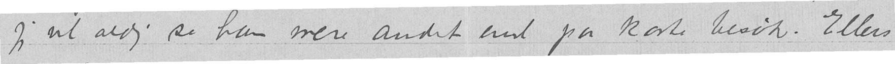jeg vel aldrig se ham mere andet end paa korte besøk. Ellers
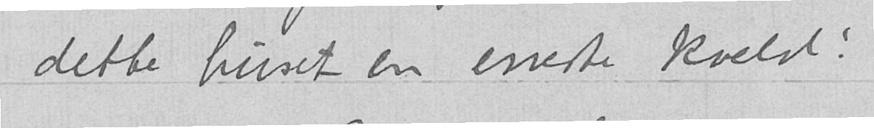dette huset en eneste kveld!
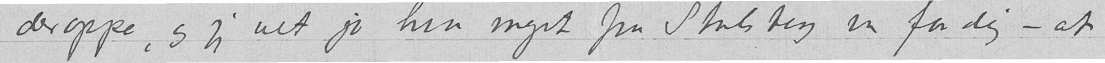deroppe, og jeg vet jo hvor meget fru Stalsberg var for dig – at
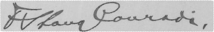F. Stang Conradi,
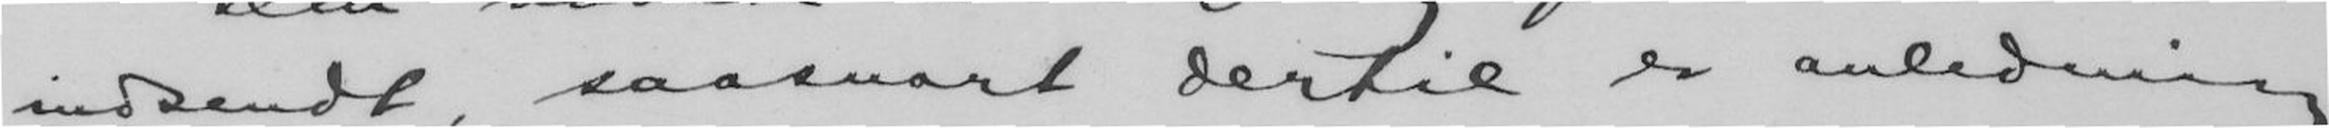indsendt, saasnart dertil er anledning.
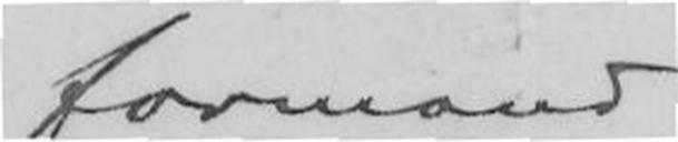formand
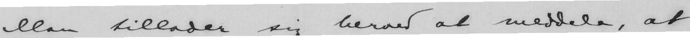Man tillader sig herved at meddele, at
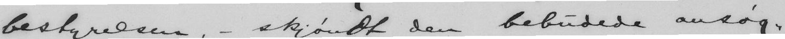bestyrelsen, - skjøndt den bebudede ansøg-
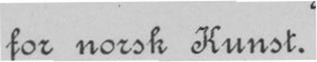for norsk Kunst.
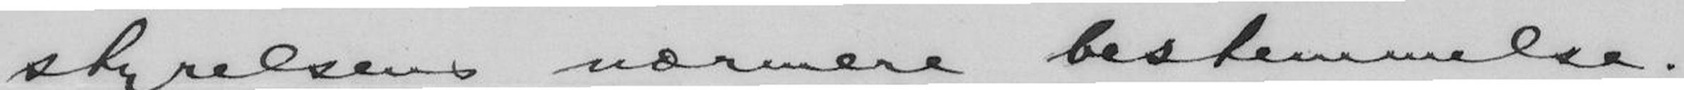styrelsens nærmere bestemmelse.
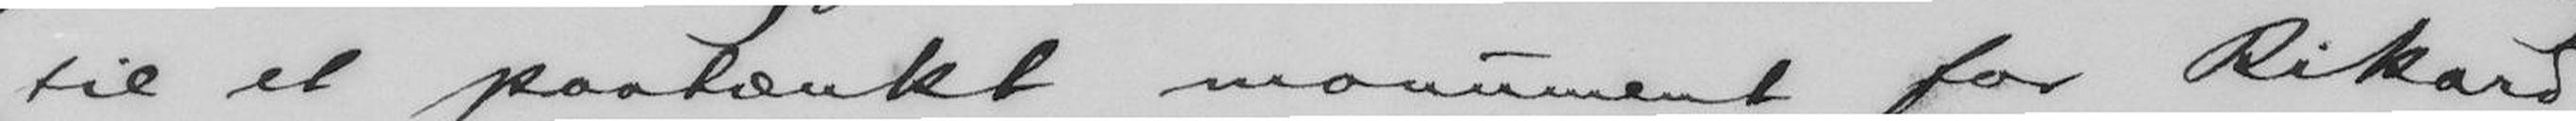til et paatænkt monument for Rikard
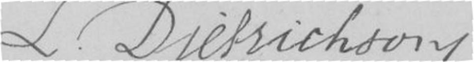L. Dietrichson
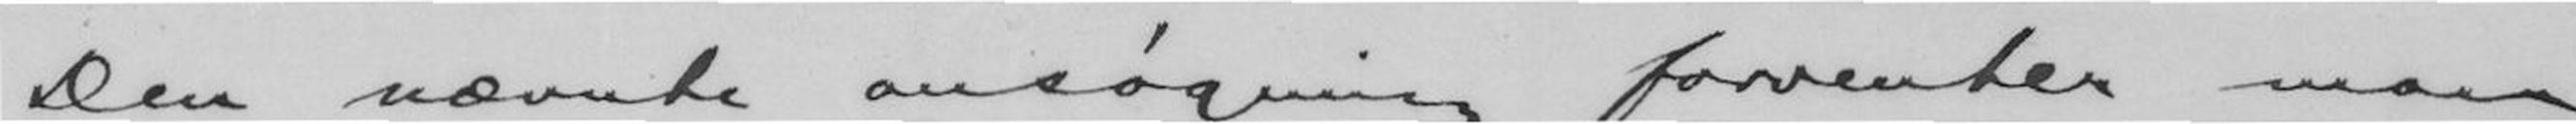Den nævnte ansøgning forventer man
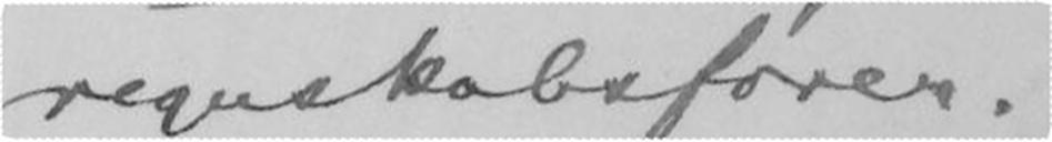regnskabsfører.
